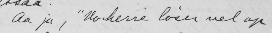Aa ja, "Vorherre løser vel op
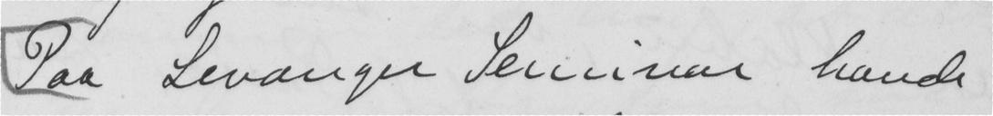Paa Levænger Seminar havde
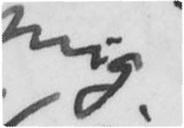mig
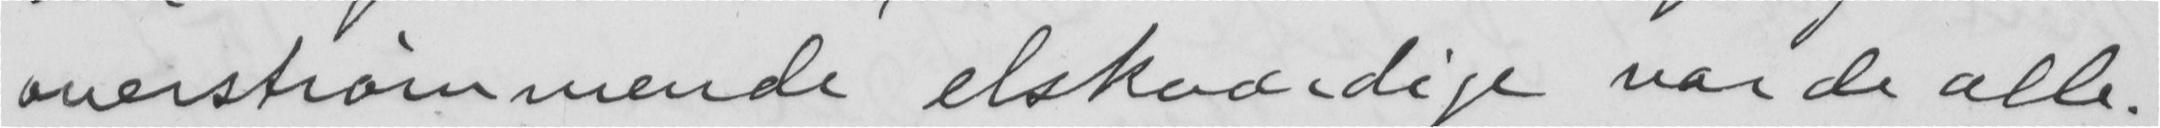overstrømmende elskværdige var de alle.
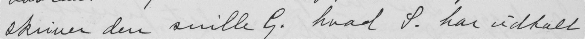skriver den snille G. hvad S. har udtalt
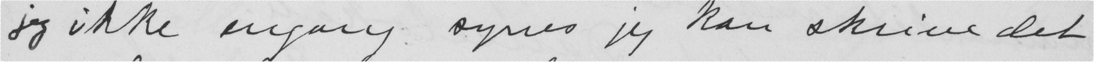jeg ikke engang synes jeg kan skrive det
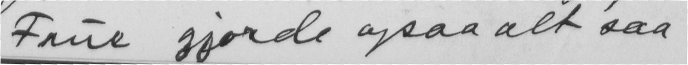Frue gjorde ogsaa alt saa
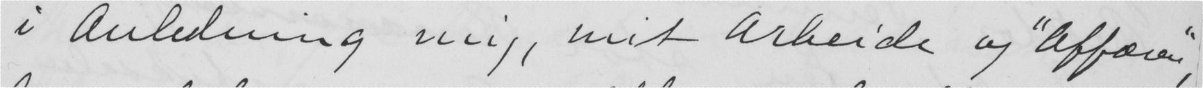i Anledning mig, mit Arbeide og "Affæren",
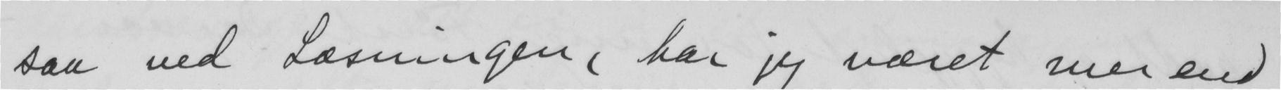saa ved Læsningen, har jeg været mer end
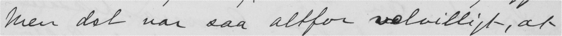Men det var saa altfor velvilligt, at
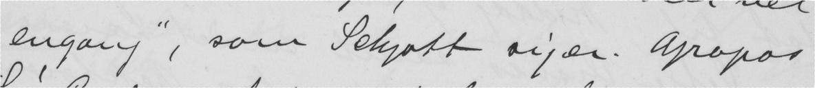engang", som Schjøtt siger. Apopos
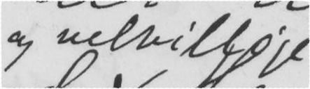og velvillige
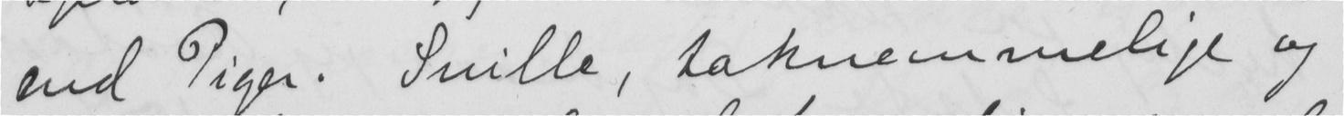end Piger. Snille, taknemmelige og
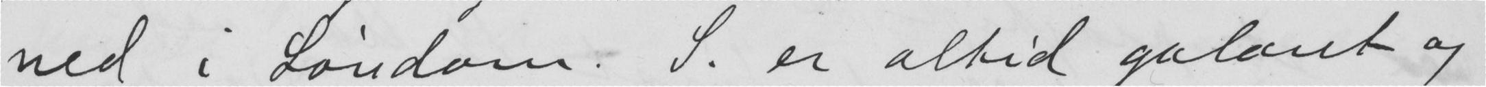ned i Løndom. S. er altid galant og
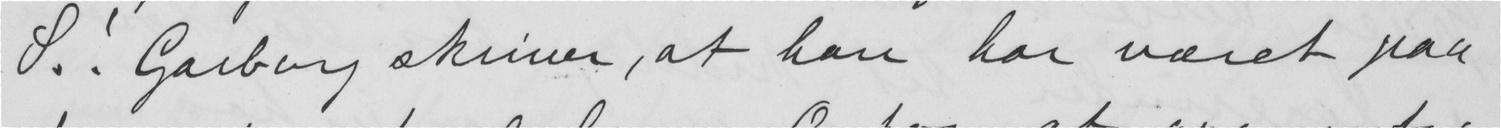S.! Garborg skriver, at han har været paa
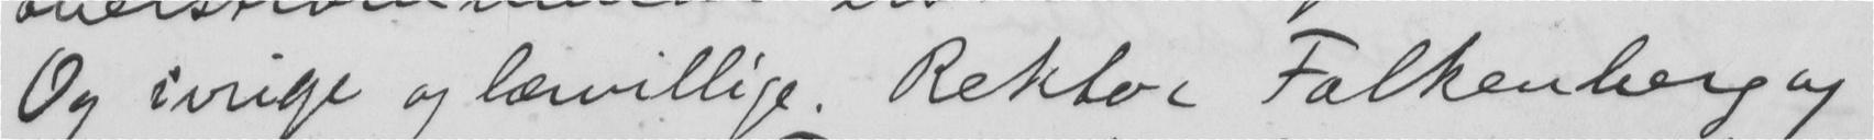Og ivrige og læsvillige. Rektor Folkenberg og
